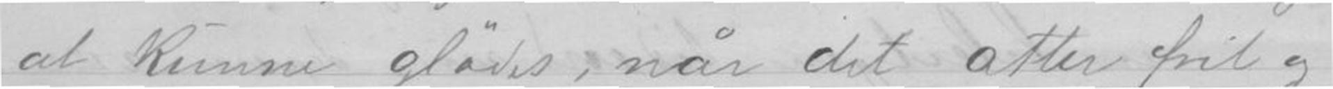at kunne glädes, når det atter frit og
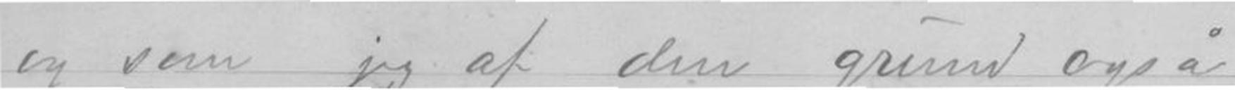og som jeg af den grund også
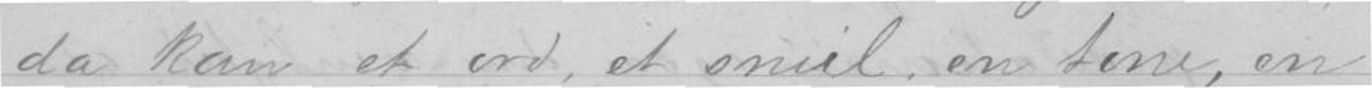da kan et ord, et smil, en tone, en
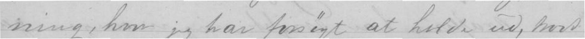ning, hvor jeg har forsøgt at holde ud, trods
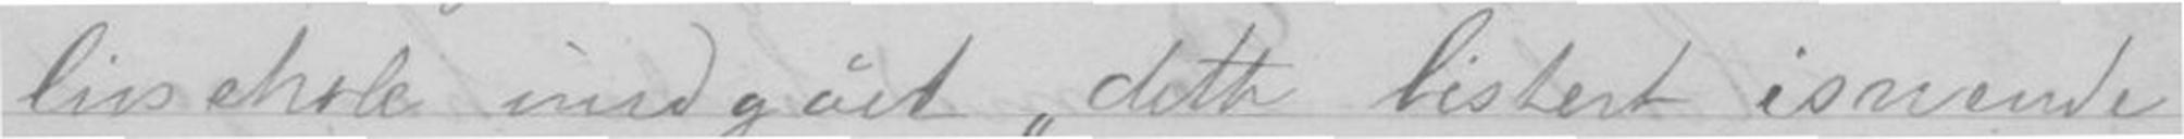livs skole undgået dette bistert isnende
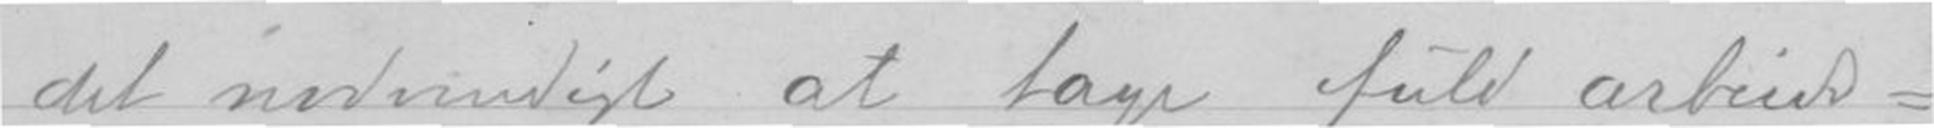det nødvendigt at tage fuld arbeids-
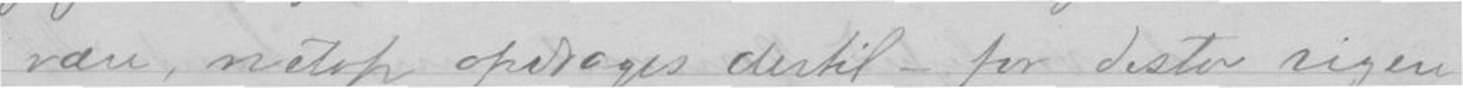være, netop opdrages dertil - for desto rigere
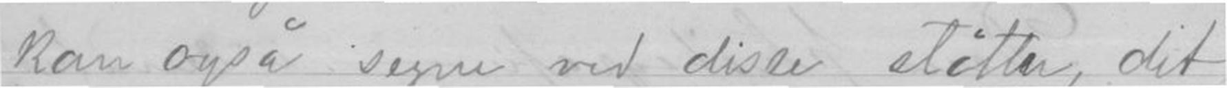kan også segne ved disse støtter, det
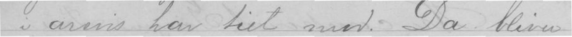i årevis har tiet med. Da bliver
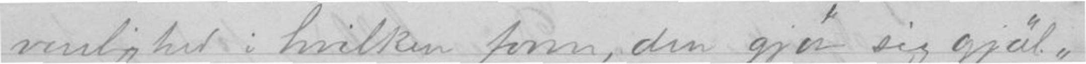venlighed i hvilken form, den gjør sig gjäl-
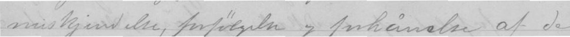miskjendelse, forfølgelse og forhånelse af de
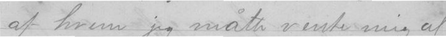af hvem jeg måtte vente mig al
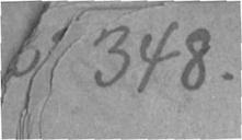348.
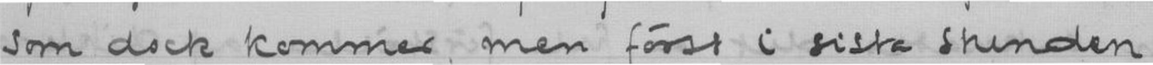som dock kommer, men först i sista stunden
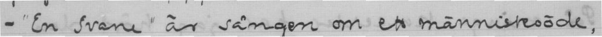- "En Svane" är sången om ett människoöde,
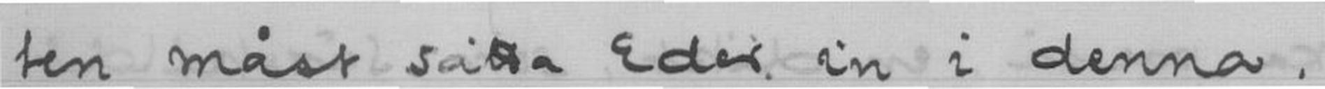ten måst sätta Eder in i denna.
